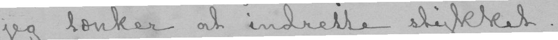jeg tænker at indrette stykket.
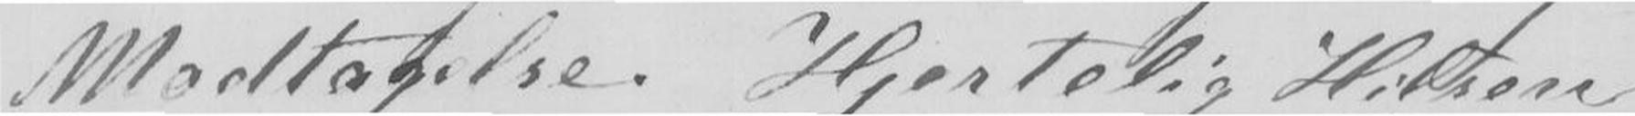Modtagelse. Hjertelig Hilsen
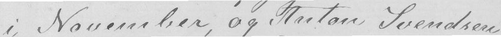i November, og Anton Svendsen
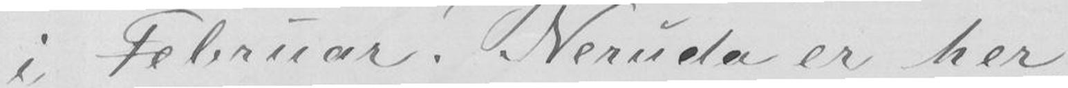i Februar. Neruda er her,
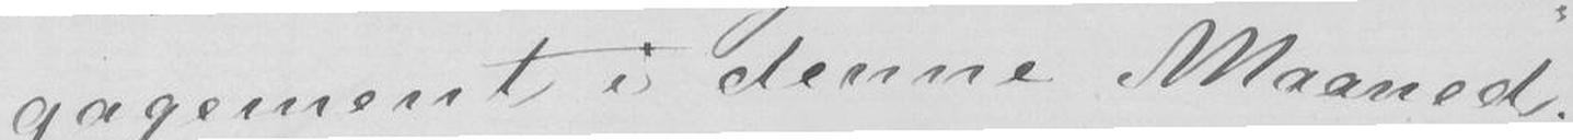gagement i denne Maaned.
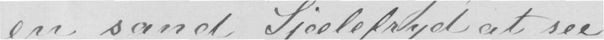en sand Sjælefryd at see
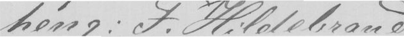heng: F. Hildebrand
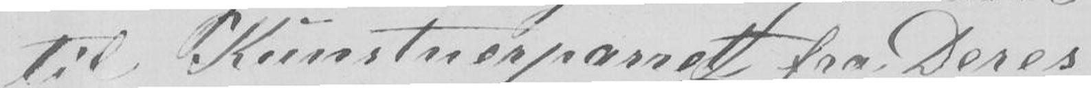til Kunstnerparret fra Deres
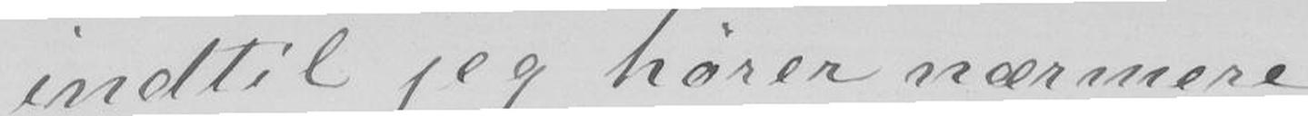indtil jeg hører nærmere
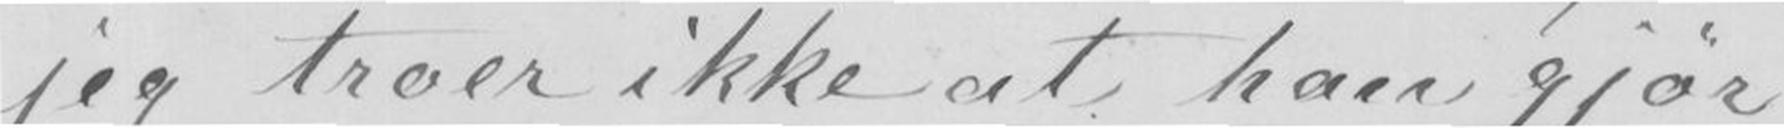jeg troer ikke at han gjør
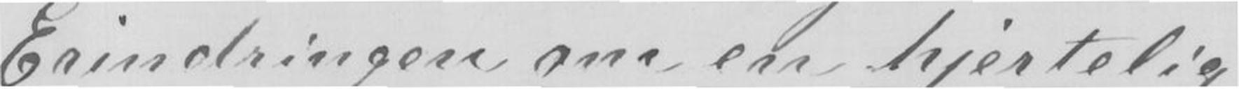Erindringen om en hjertelig
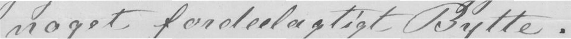noget fordeelagtigt Bytte.
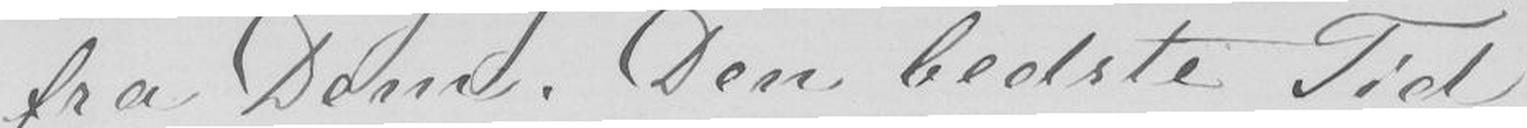fra Dem. Den bedste Tid
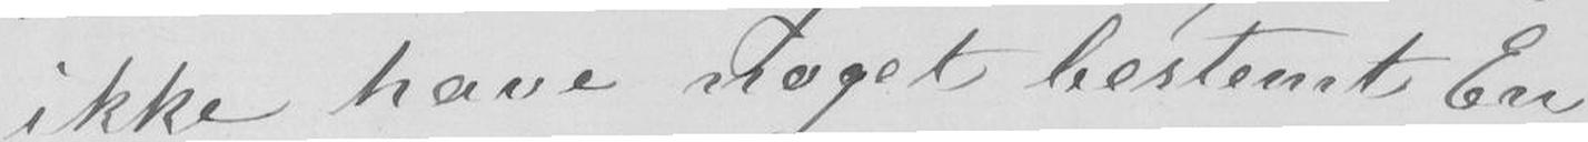ikke have noget bestemt En-
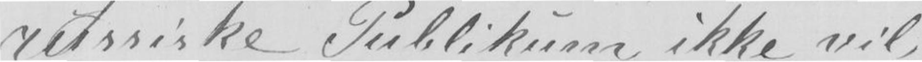russiske Publikum ikke vil
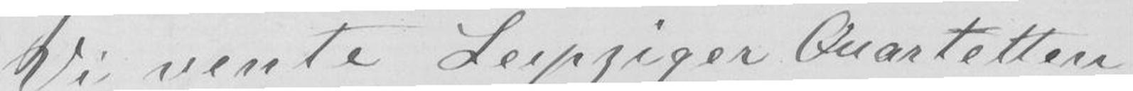Vi vente Leipziger Quartetten
